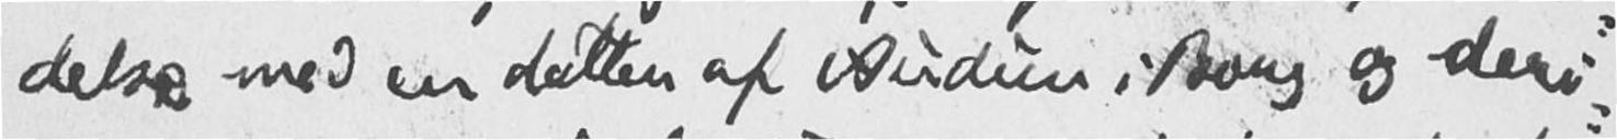delse med en datter af Audun Borg og deri-
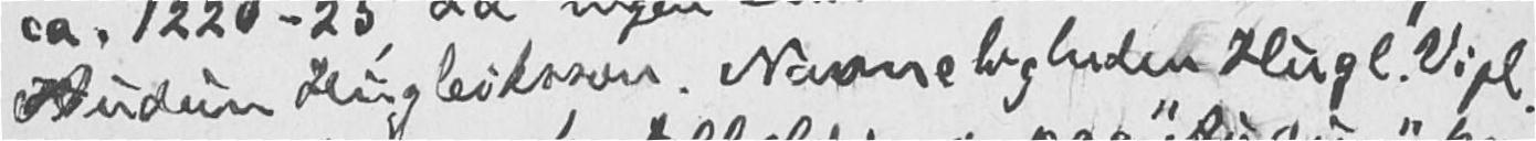Audun Hugleiksson. Navneligheden Hugl. Vigl.
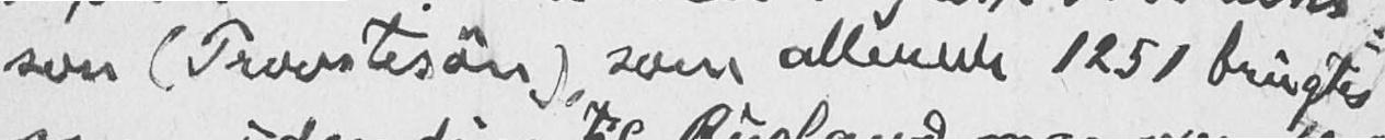son (Provstesøn), som allerede 1251 brugtes
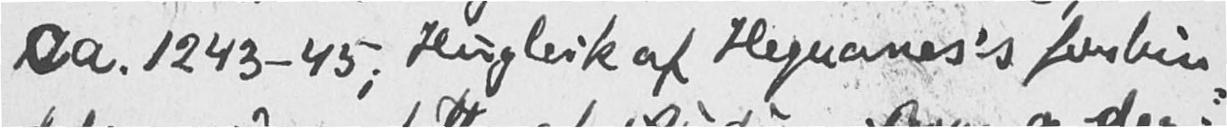ca. 1243-45; Hugleik af Hegranes's forbin-
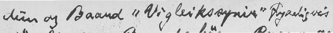dun og Baard "Vigleikssynir" tydeligvis
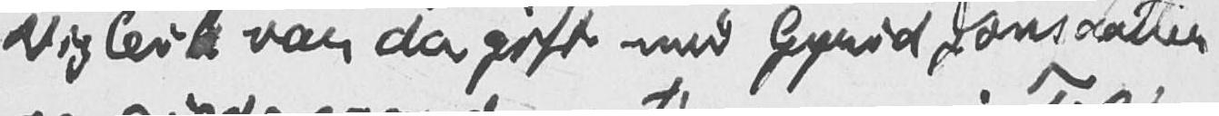Vigleik var da gift med Gyrid Jonsdatter
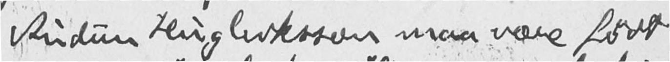Audun Hugleiksson maa være født
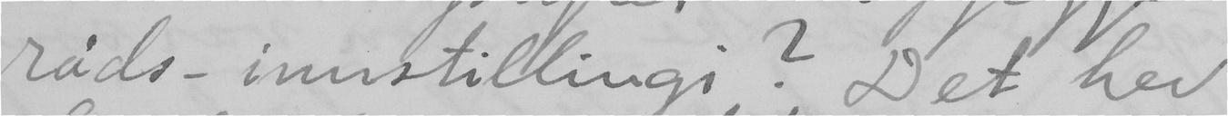råds-innstillingi? Det hev
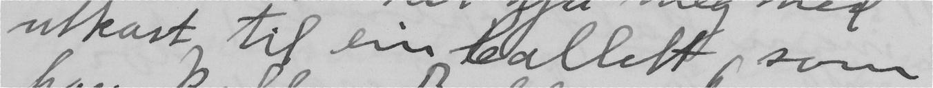utkast til ein ballett, som
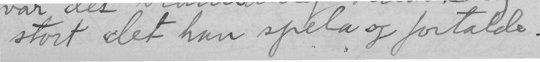stort det han spela og fortalde.
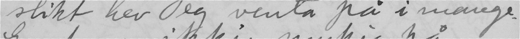slikt hev eg venta på i mange.
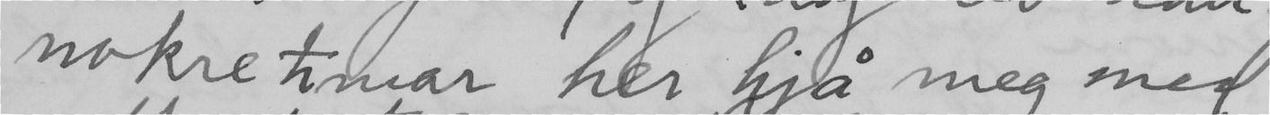nokre timar her hjå meg med
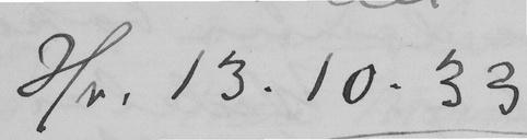Hv. 13.10.33
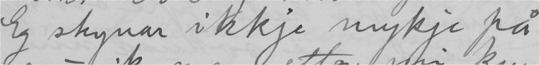Eg skynar ikkje mykje på
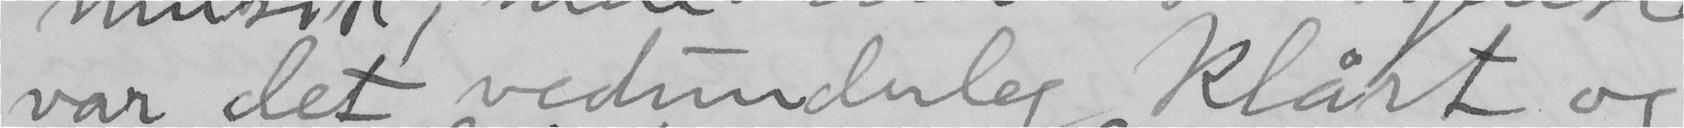var det vedunderleg klårt og
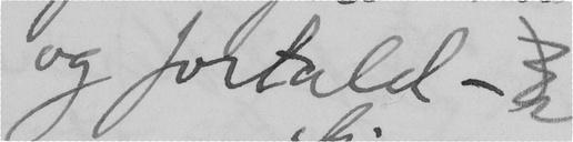og fortald –
